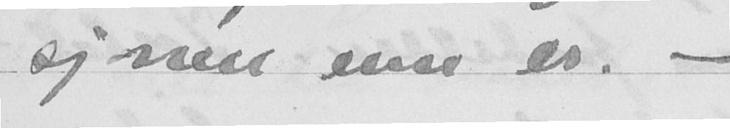sjansen enn er. -
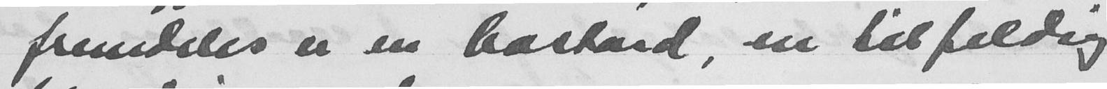fremdeles er en bastard, en tilfeldig
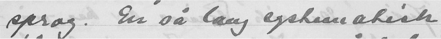sprog. En så lang systematisk
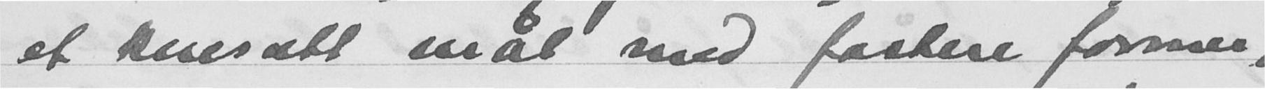et knesatt mål med fastere former,
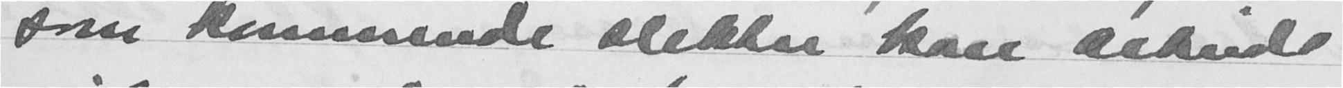som kommende slekter kan arbeide
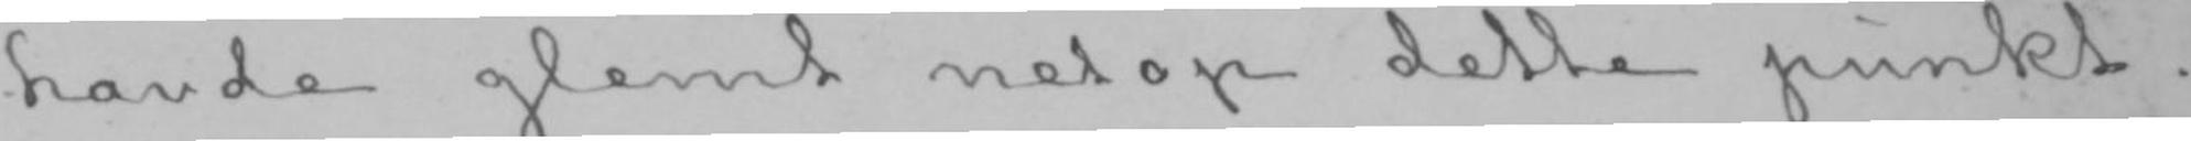havde glemt netop dette punkt.
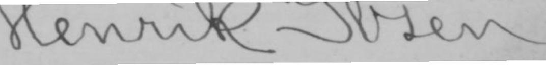Henrik Ibsen.
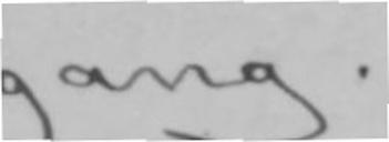gang.
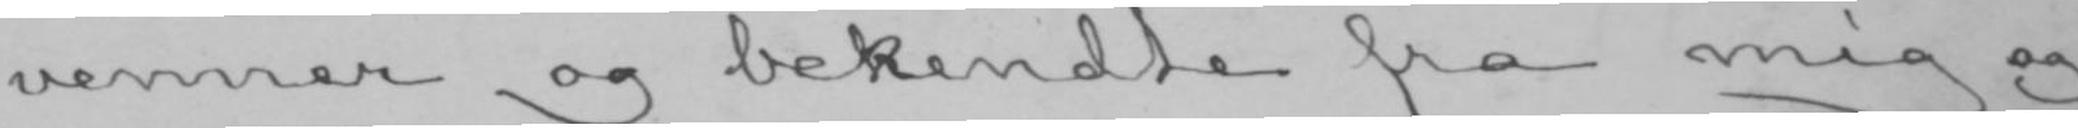venner og bekendte fra mig og
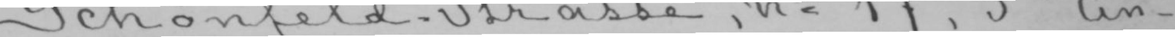Schönfeld-Strasse, no 17, 3ten Ein-
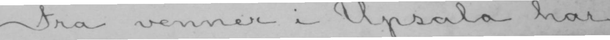Fra venner i Upsala har
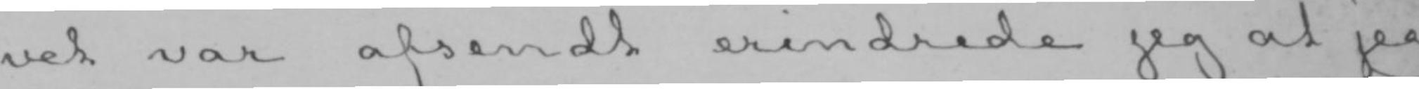vet var afsendt erindrede jeg at jeg
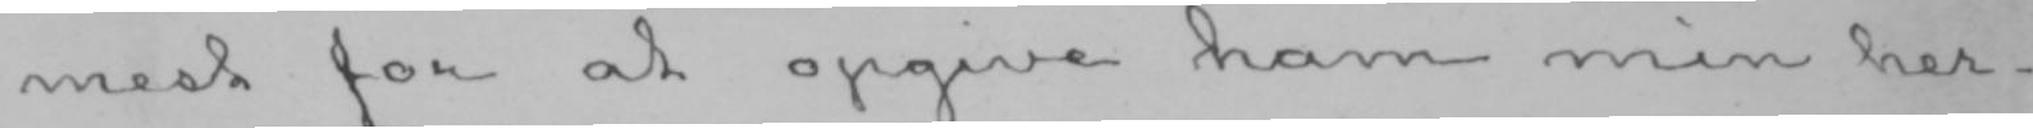mest for at opgive ham min her-
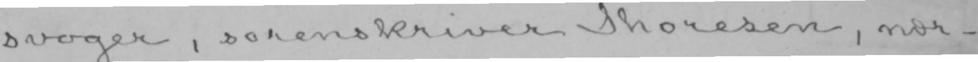svoger, sorenskriver Thoresen, nær-
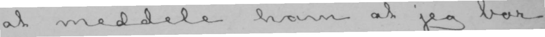at meddele ham at jeg bor
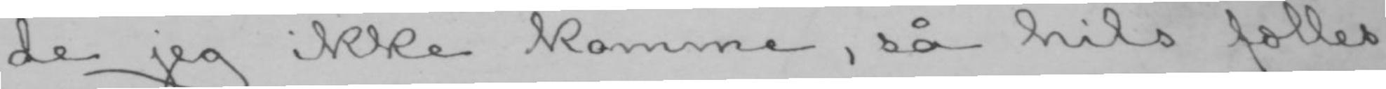de jeg ikke komme, så hils fælles
